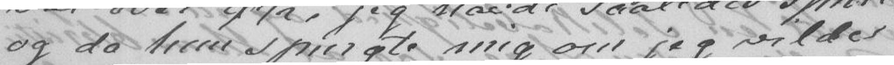og da hun spurgte mig om jeg vilde
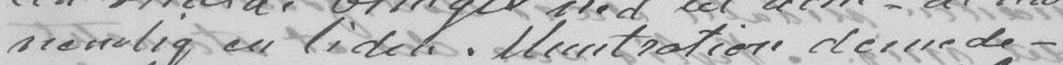nemlig en liden Muntration dernede -
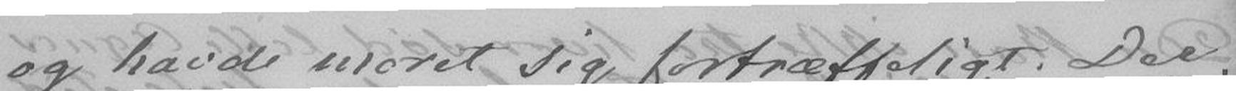og havde moret sig fortræffeligt. Der
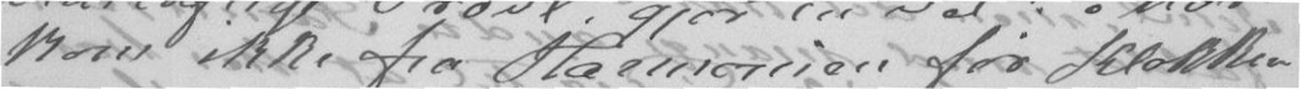kom ikke fra Harmonien før Klokken
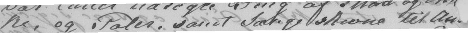ke, og Taler samt Sange skrevne til An-
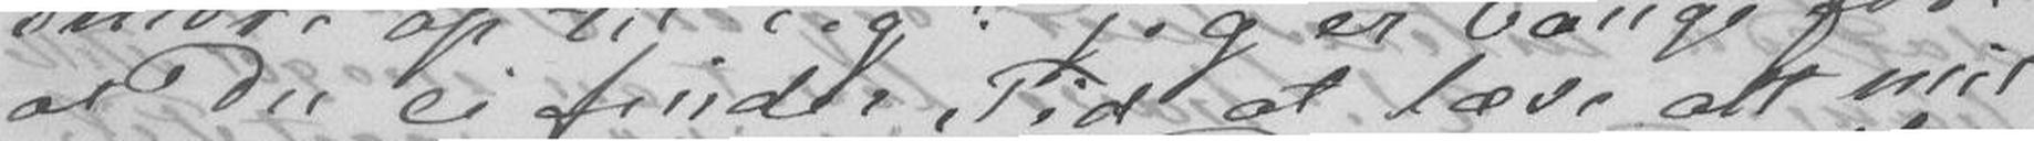at Du ei finder Tid at læse alt mit
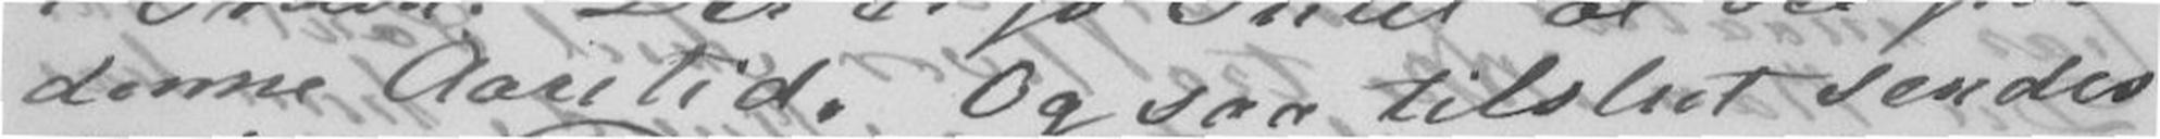denne Aarstid. Og saa tilslut sendes
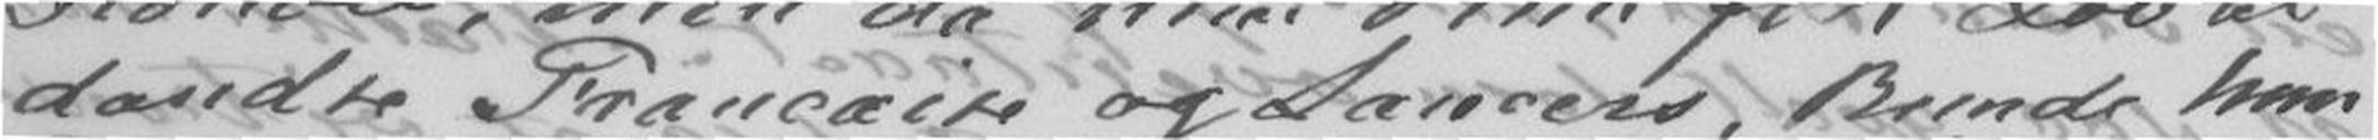dandse Francaire og Lancers, kunde hun
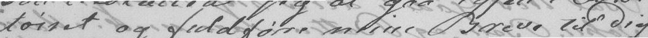toiret og fuldføre mine Breve til Dig
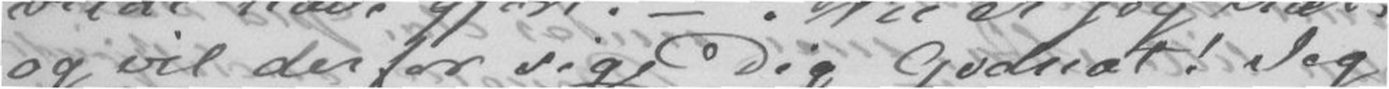og vil derfor sige Dig Godnat! Jeg
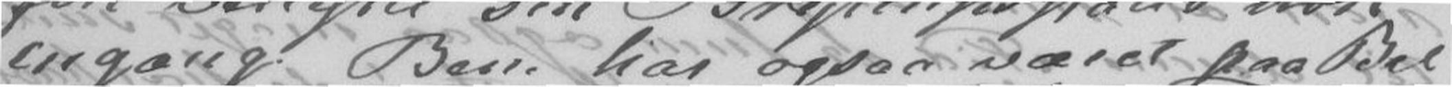engang. Bene har ogsaa været pa Bal
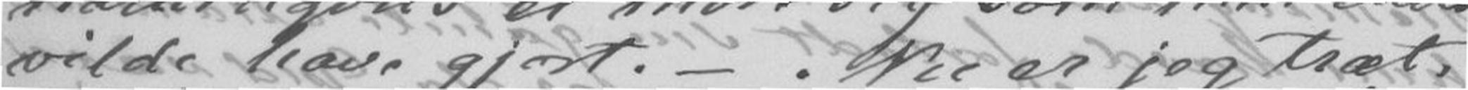vilde have gjort. - Nu er jeg træt,
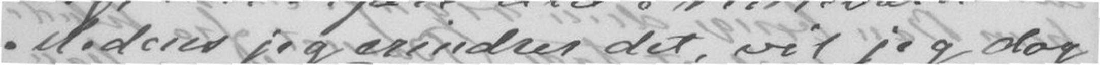Medens jeg erindrer det, vil jeg dog
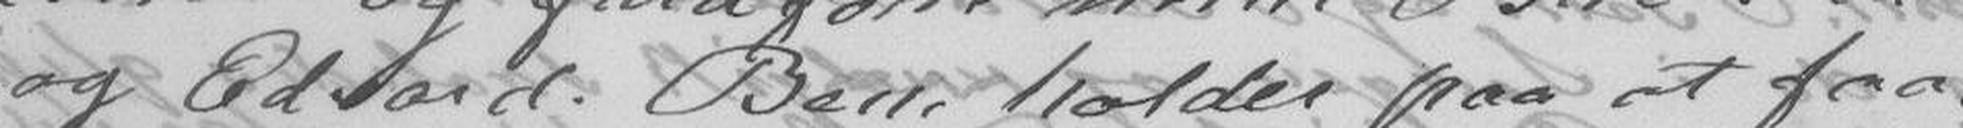og Edvard. Bene holder paa at faa
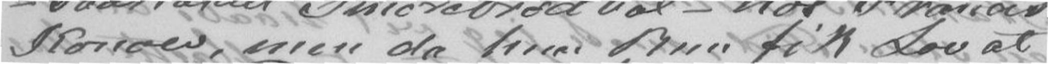Konow, men da hun kun fik Lov at
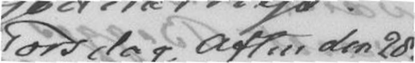Torsdag Aften den 28de
